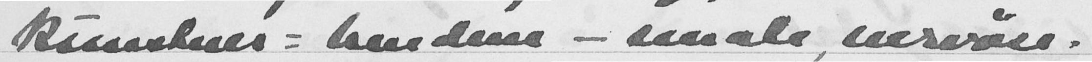kamstues-hendene - smale, nervøse.
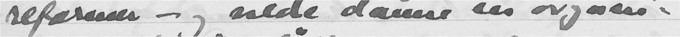reformer - og vilde danne en organi-
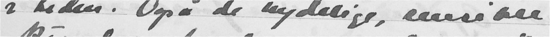i tiden. Også de nydelige, sensible
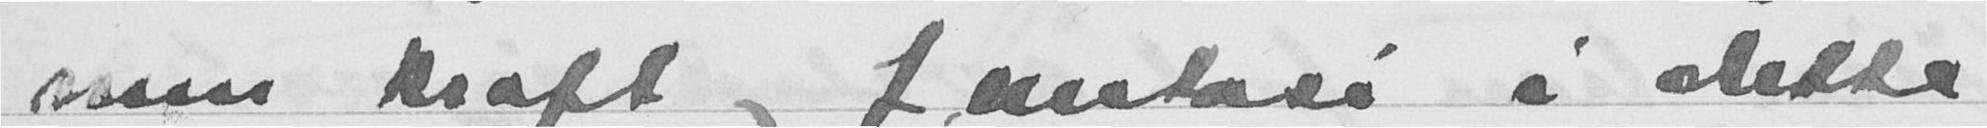men kraft og fantasi i dette
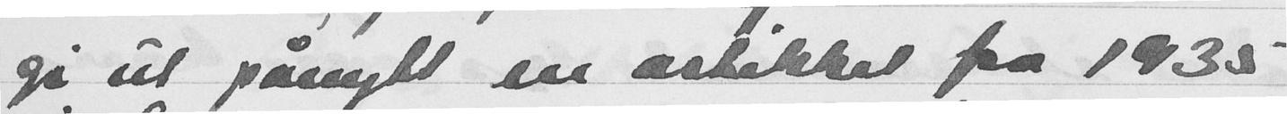gi ut pånytt en artikkel fra 1935
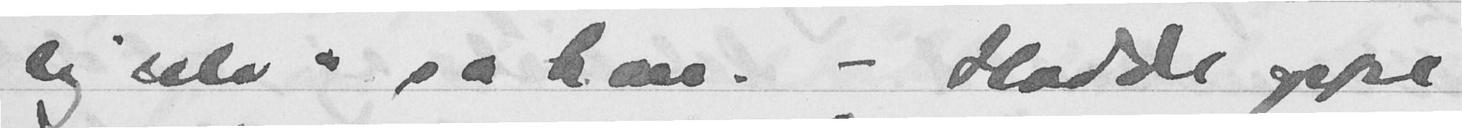sig selv" sa han. - Hadde oppe
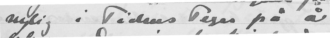nylig i Tidens Tegn på å
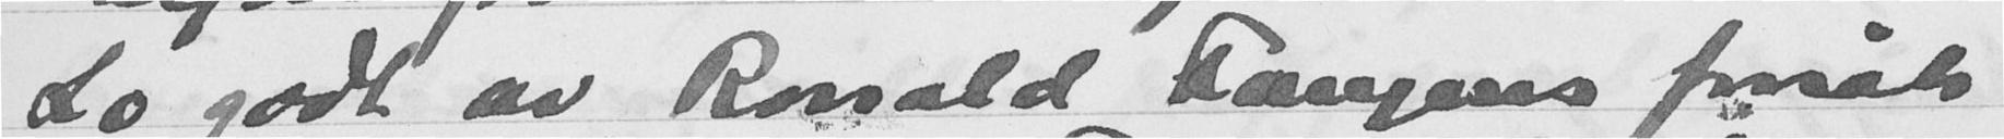Lo godt av Ronald Fangens forsøk
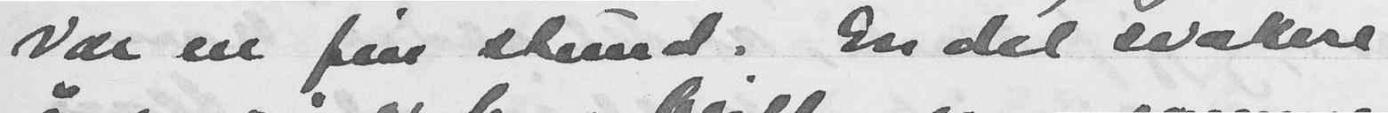var en fin stund. En del svakere
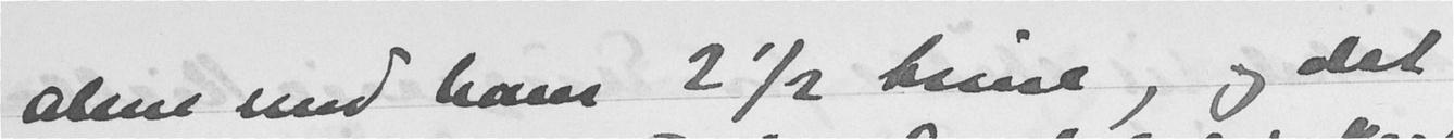alene med ham 2 ½ time, og det
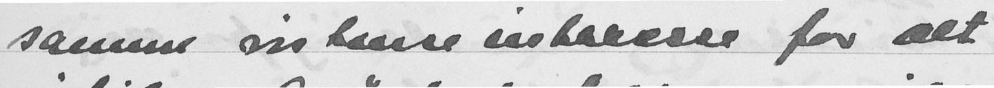samme intense interesse for alt
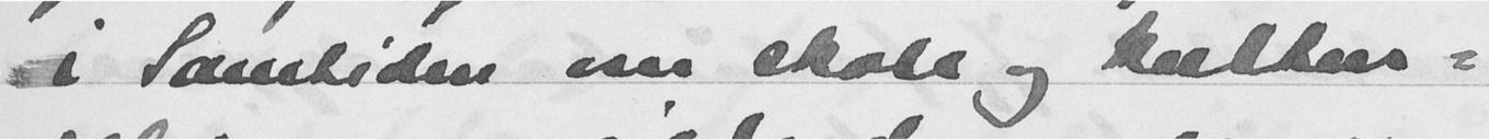i Samtiden om skole og kultur-
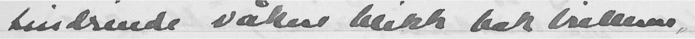tindrende våkne blikk bak brillene,
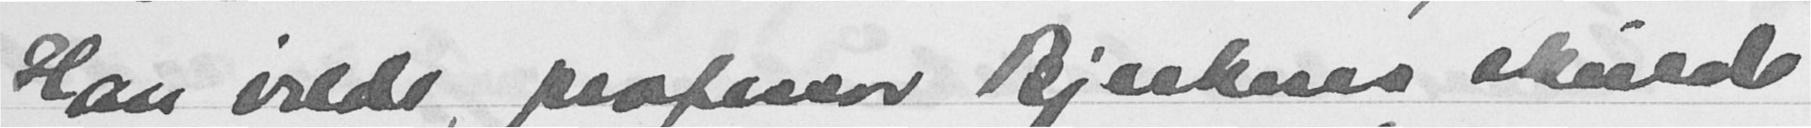Han vilde, professor Bjerknes skulde
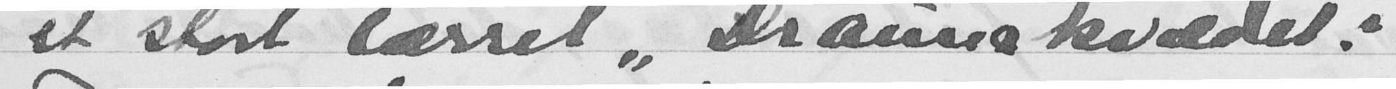et stort lærret "Draumekvædet"
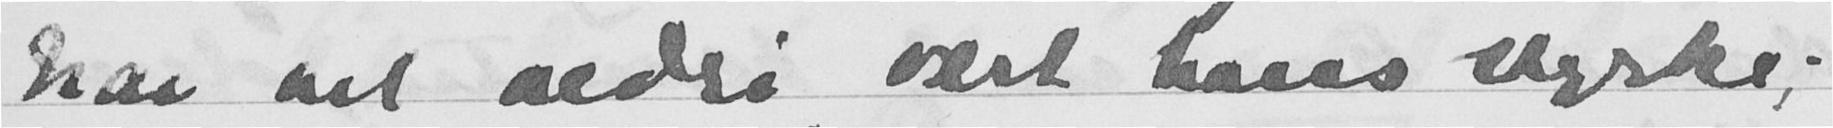har vel aldri vært hans styrke;
